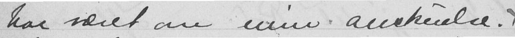har været om min anskuelse. x
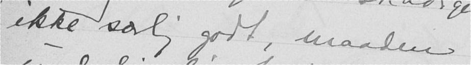ikke særlig godt, maaden
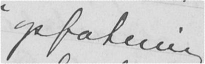opfatning
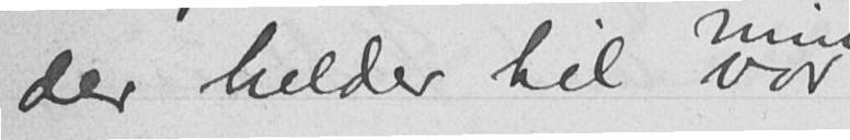der holder til vor
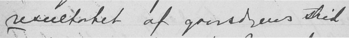resultatet af gaarsdagens strid
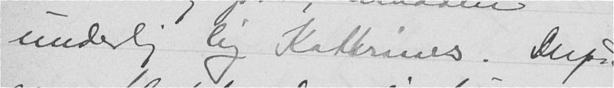underlig lig Kathrines. Derpaa
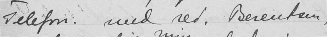Telefon. med red. Berentsen,
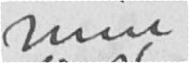min
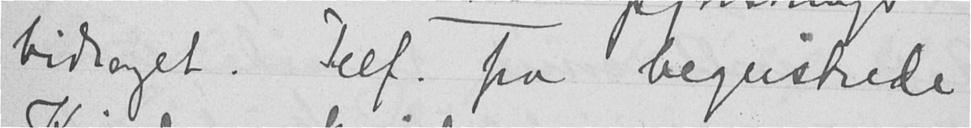bidraget. Telf. fra begeistrede
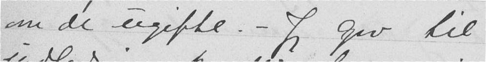om de ugifte. - Jeg gav til
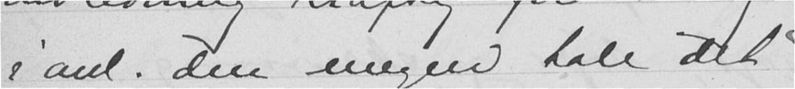i anl. den megen tale det
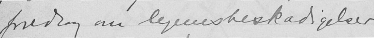foredrag om legemsbeskadigelser
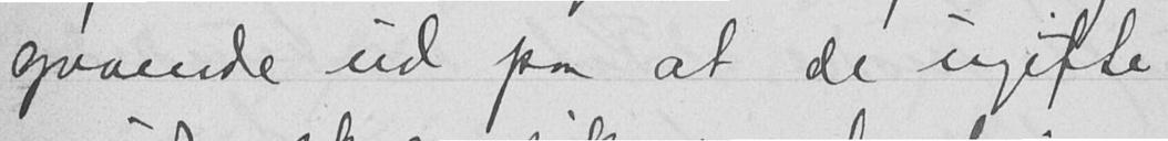gaaende ud paa at de ugifte
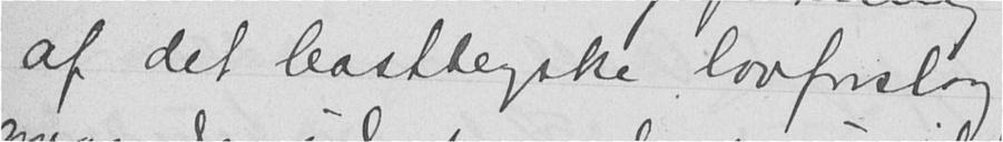af det Castbergske lovforslag
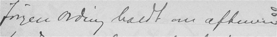Jørgen Ording holdt om aftenen
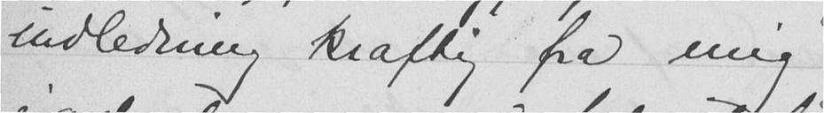indledning kraftig fra mig
